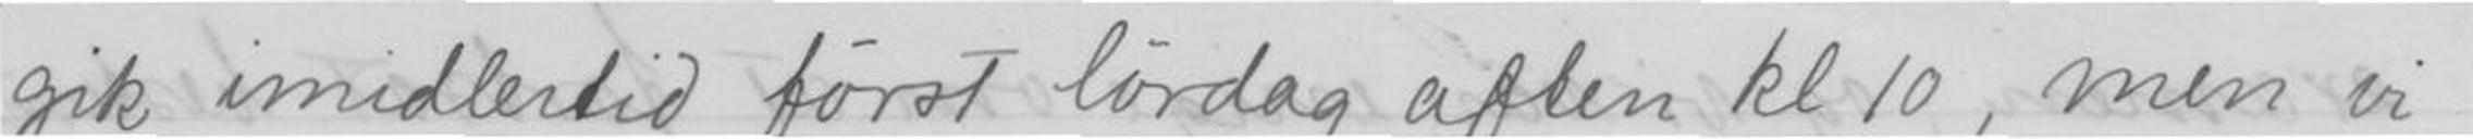gik imidlertid først lørdag aften kl 10, men vi
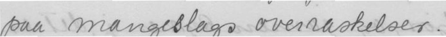paa mangeslags overraskelser.
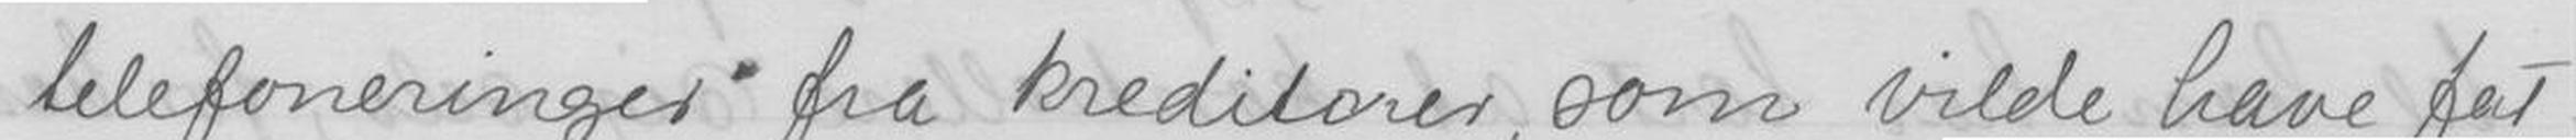telefoneringer fra kreditorer, som vilde have fat
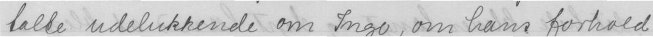talte udelukkende om Ingo, om hans forhold
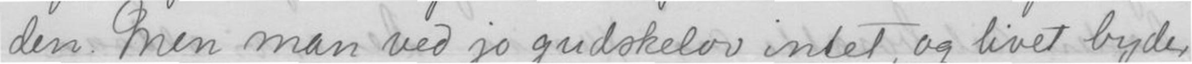den. Men man ved jo gudskelov intet, og livet byder
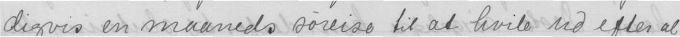digvis en maaneds søreise til at hvile ud efter al
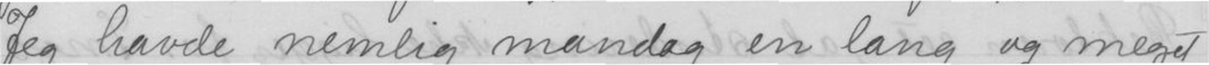Jeg havde nemlig mandag en lang og meget
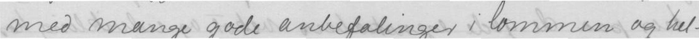med mange gode anbefalinger i lommen og hel-
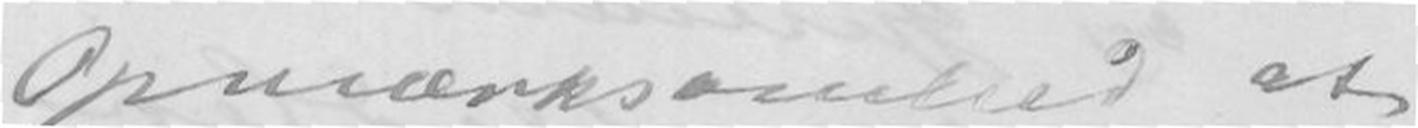opmærksomhed at
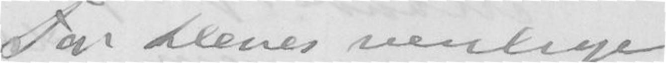For Deres venlige
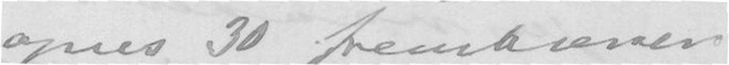opus 30 fremkrever
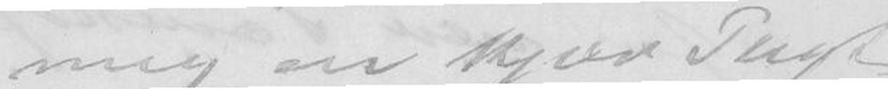mig en kjær Pligt
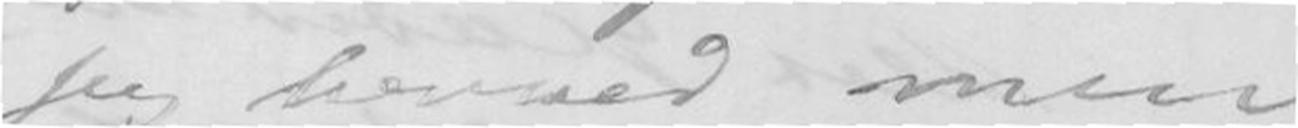jeg herved min
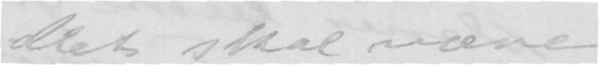Det skal være
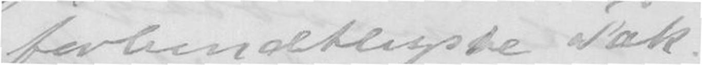forbindtligste Tak.
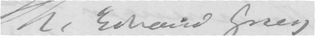Hr Edvard Grieg
